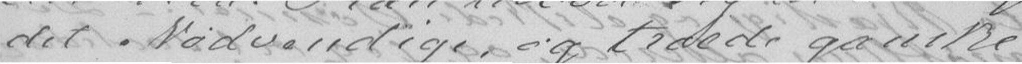det Nødvendige, og troede ganske
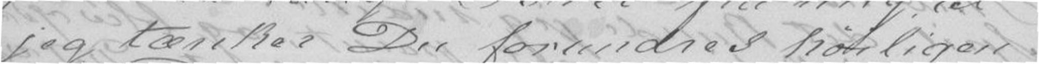jeg tænker Du forundres høiligen
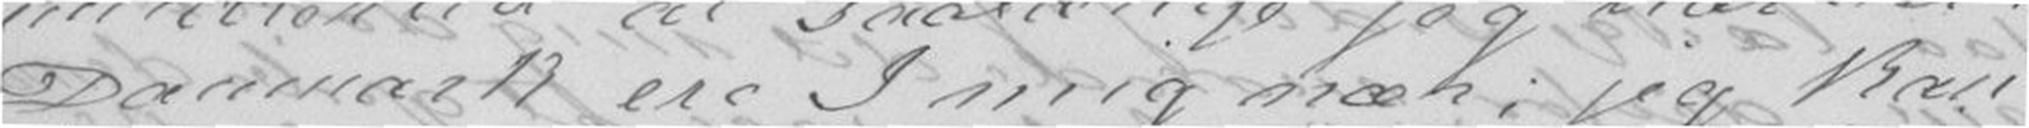Danmark ere I mig nær; jeg kan
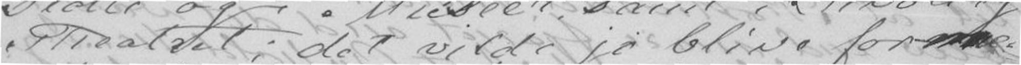Thatret; det vilde jo blive for me-
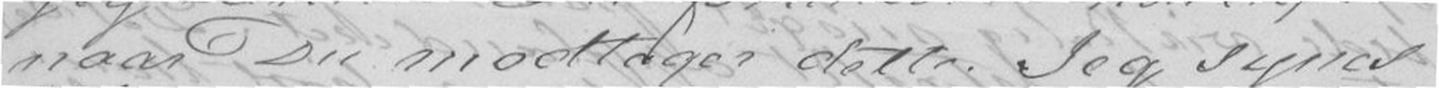naar Du modtager dette. Jeg synes
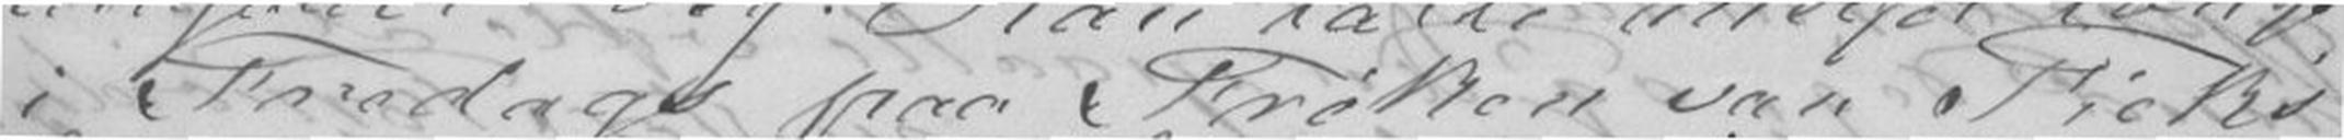i Fredags paa Frøken von Ficks
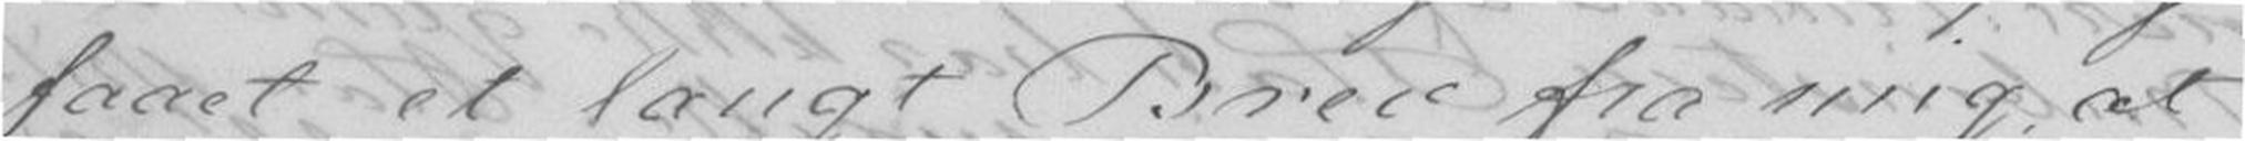faaet et langt Breve fra mig at
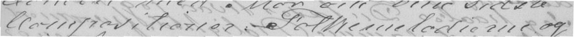Compositioner. Folkemelodierne og
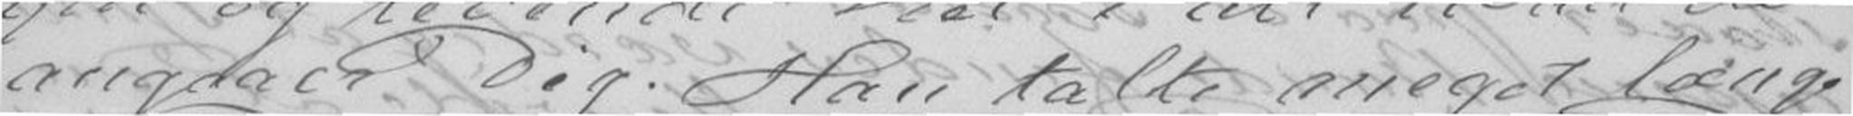angaar Dig. Han talte meget længe
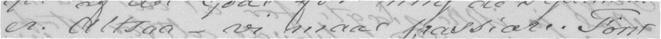er. Altsaa - vi maae passiare. Først
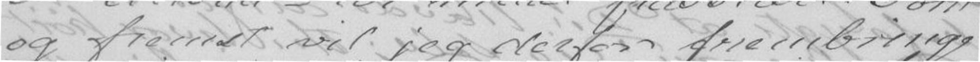og fremst vil jeg derfor frembringe
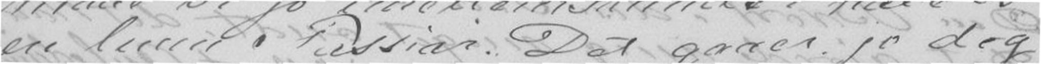en liden Passiar. Det gaaer jo dog
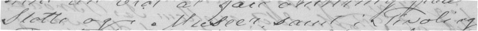Slotte og Museer, samt i Tivoli og
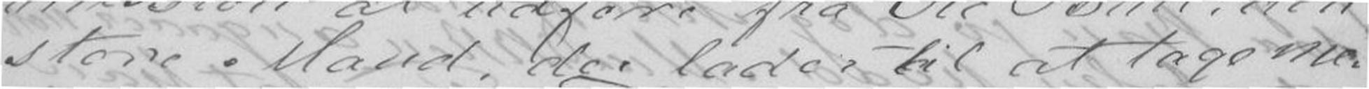store Mand, der lader til at tage me-
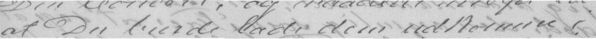at Du burde lade dem udkomme i
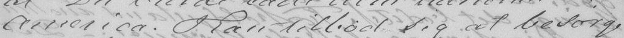America. Han tilbød sig at besørge
